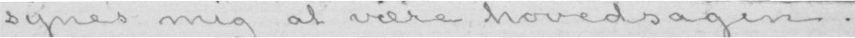synes mig at være hovedsagen.
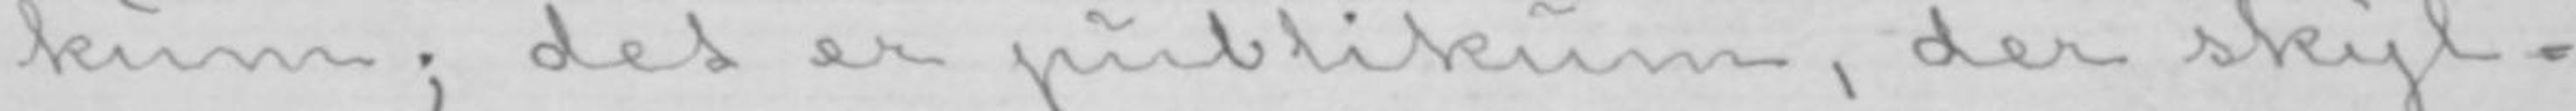kum; det er publikum, der skyl-
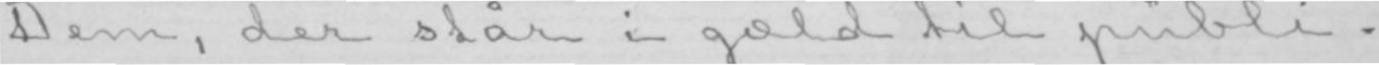Dem, der står i gæld til publi-
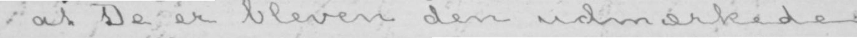at De er bleven den udmærkede
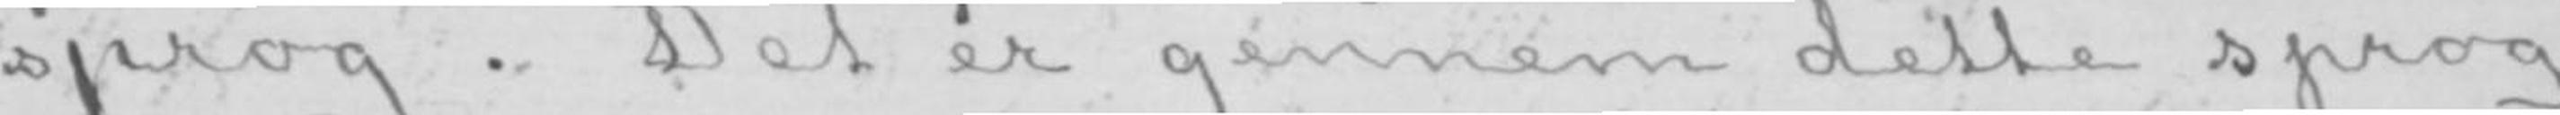sprog. Det er gennem dette sprog
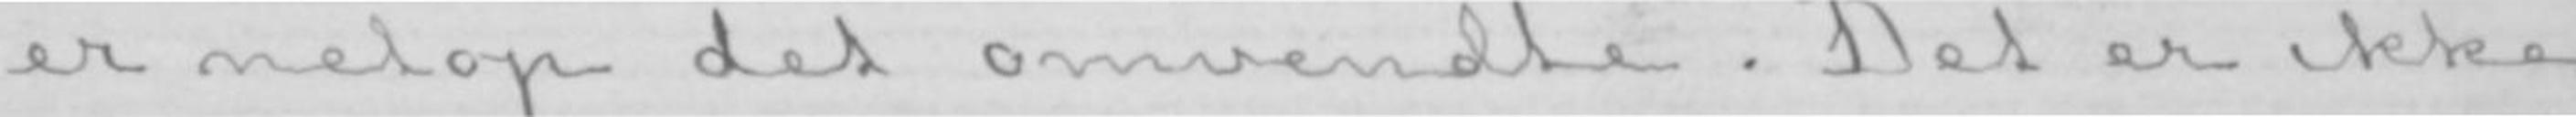er netop det omvendte. Det er ikke
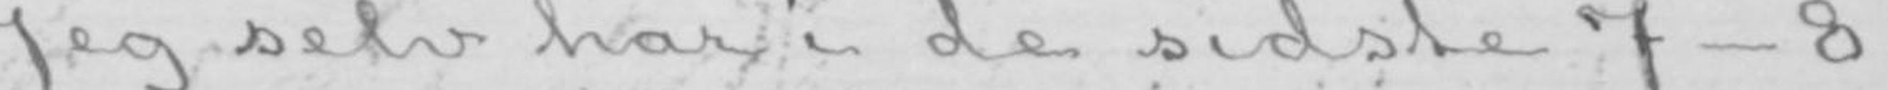Jeg selv har i de sidste 7-8
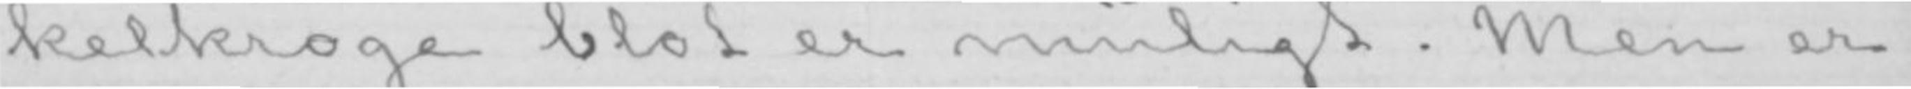kelkroge blot er muligt. Men er
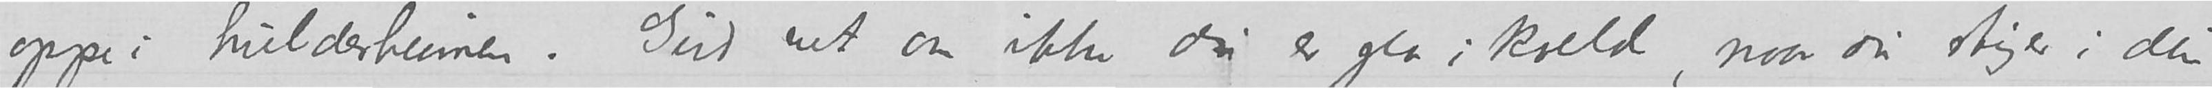oppe i hulderheimen. Gud vet om ikke du er gla ikveld , naar du stiger i din
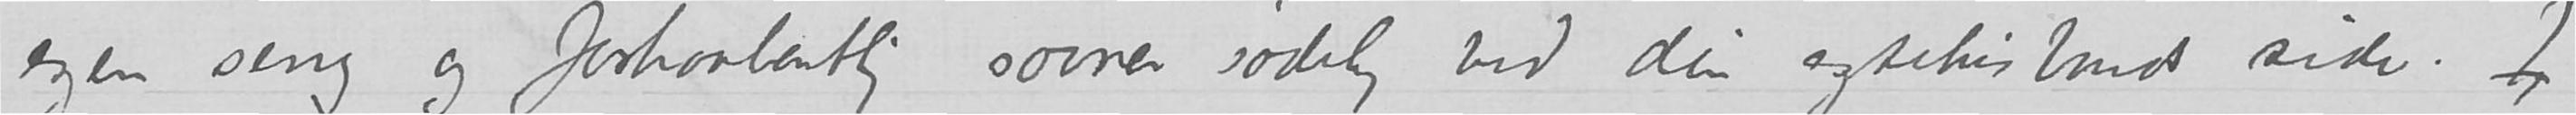egen seng og forhaabentlig sovner sødelig ved din ægtehusbonds side. Jeg
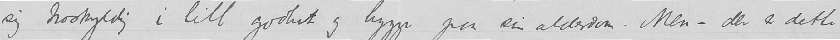soler hun sig troskyldig i litt godhet og hygge paa sin alderdom. Men – der er dette
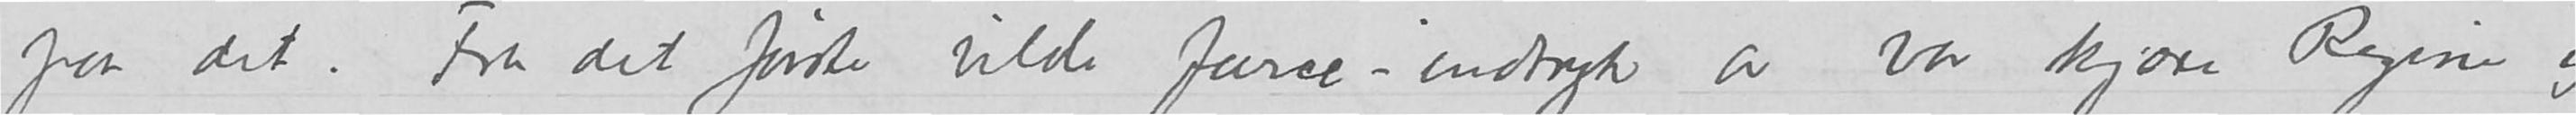paa det. Fra det første vilde farce-indtryk av voaar kjære Regine og
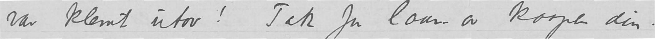var klemt utav! Tak for laan av kaapen din.
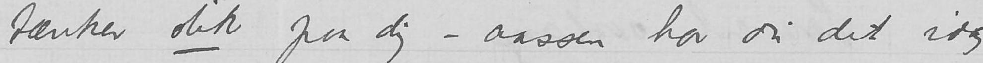Jeg tænker slik på dig – aassen har du det idag
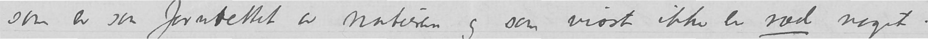som er saa forutrettet av naturen og som visst ikke er ræd noget.
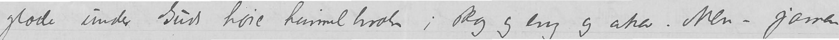glæde under Guds høie himmelhvælv i skog og eng og aker.  Men – jamen
